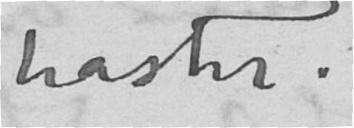haster.
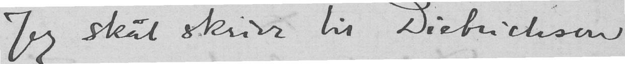Jeg skal skrive til Dietrichson
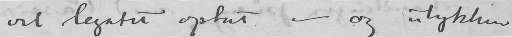vel legatet optat - og ulykken
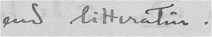end litteratur.
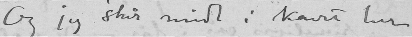Og jeg står midt i kavet her
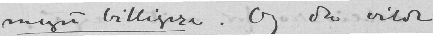meget billigere. Og da vilde
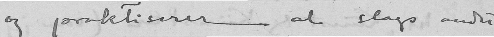og praktiserer al slags andet
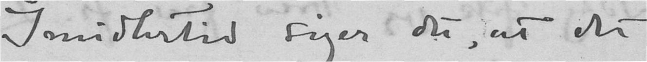Imidlertid siger du, at det
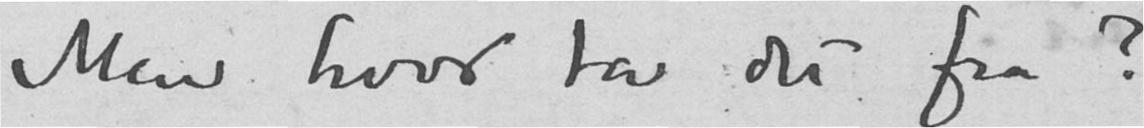Men hvor ta det fra?
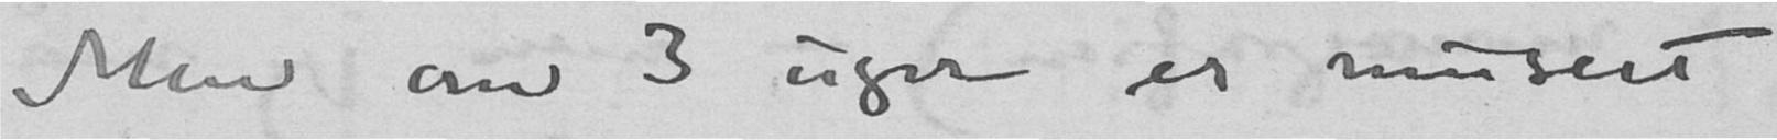Men om 3 uger er museet
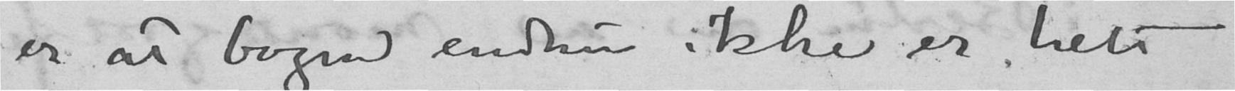er at bogen endnu ikke er helt
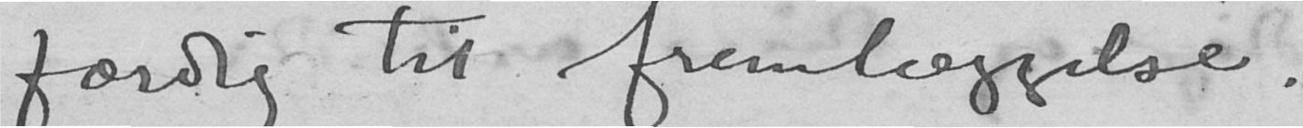færdig til fremlæggelse.
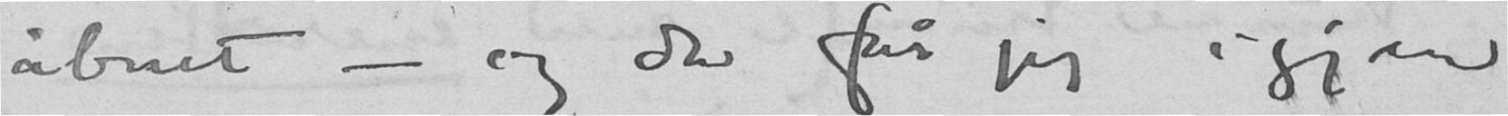åbnet - og da får jeg igjen
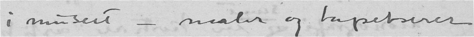i museet - maler og tapetserer
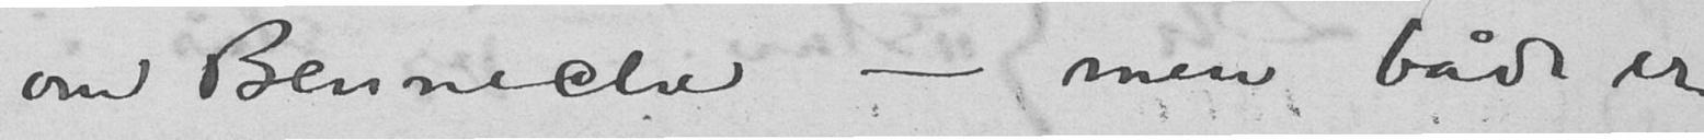om Benneche - men både er
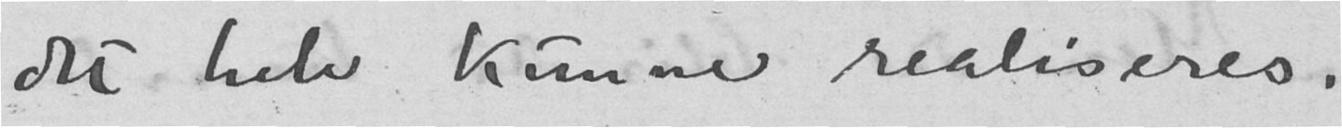det hele kunne realiseres.
